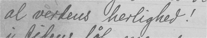al verdens herlighed!
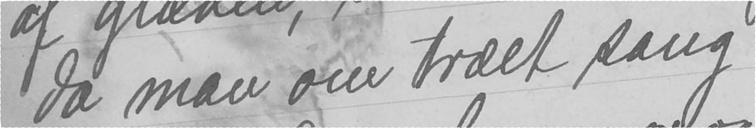da man om træet sang
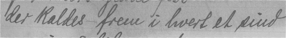der kaldes frem i hvert et sind
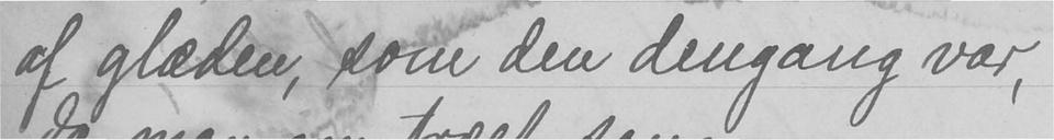af glæden, som den dengang var,
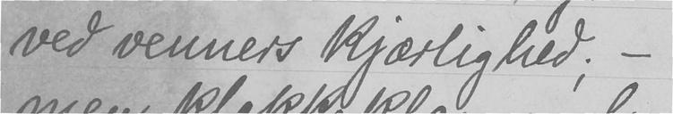ved venners kjærlighed; -
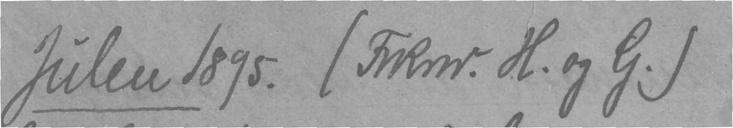Julen 1895. (Frknr. H. og G.)
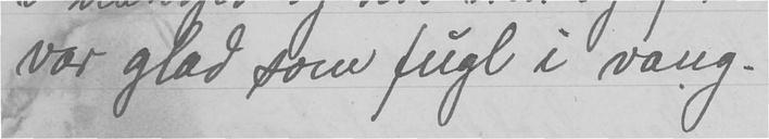var glad som fugl i vang.
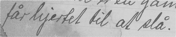- får hjertet til at slå.
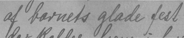af barnets glade fest
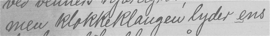men klokkeklangen lyder ens
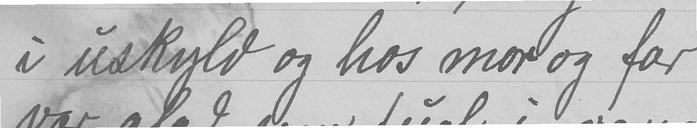i uskyld og hos mor og far
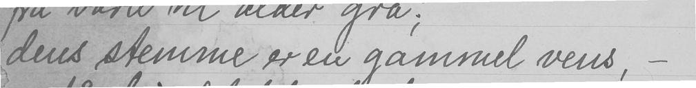dens stemme er en gammel vens, -
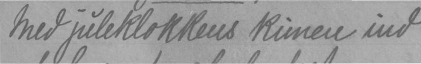Med jueleklokkens kimen ind
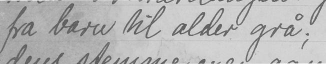fra barn til alder grå;
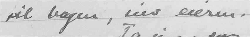til byen, sier eieren.
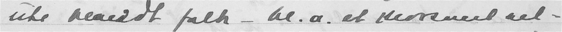ute blandt folk - bl. a. et morsomt sel-
